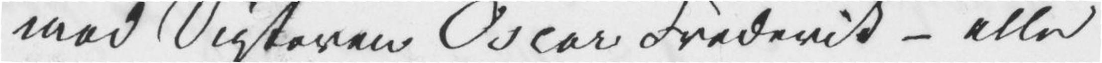mod Digteren Oscar Frederik – eller
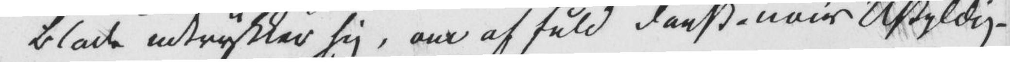Blade udtrykker sig, om af fuld dansk naiv Uskyldig¬
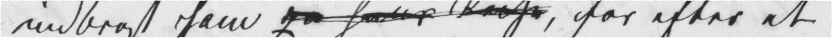indbragt ham på hans Reise, for efter et
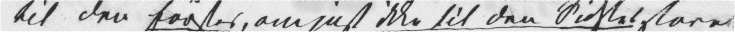til den førstes, om just ikke til den Sidstes store
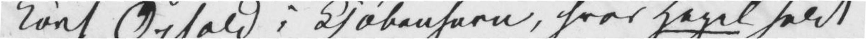kort Ophold i Kjøbenhavn, hvor Hegel holdt
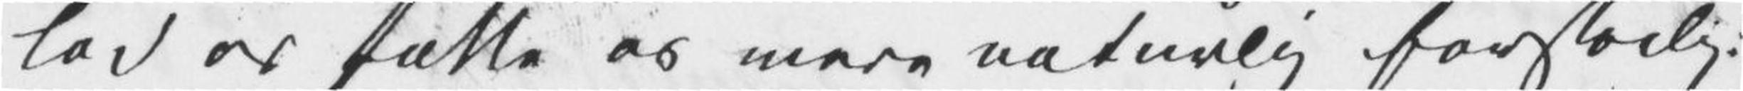lad os fatte os mere naturlig forståelig:
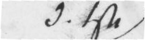d. 1ste
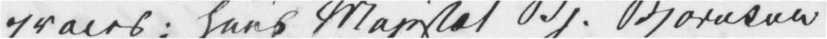proces: Hans Majestæt Bj. Bjørnson
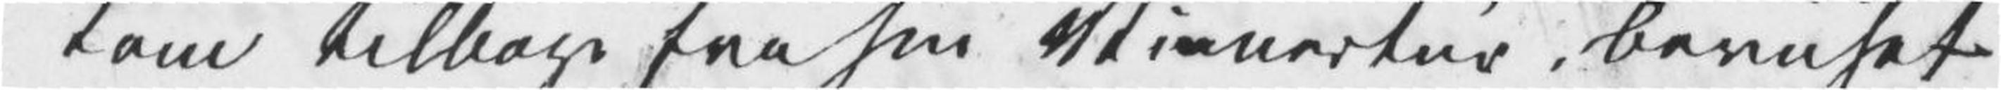kom tilbage fra sin Wienertur, beruset
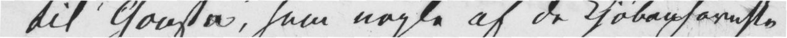til «Gousla», som nogle af de Kjøbenhavnske
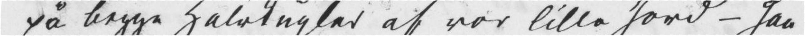på begge Halvkugler af vor lille Jord – Han
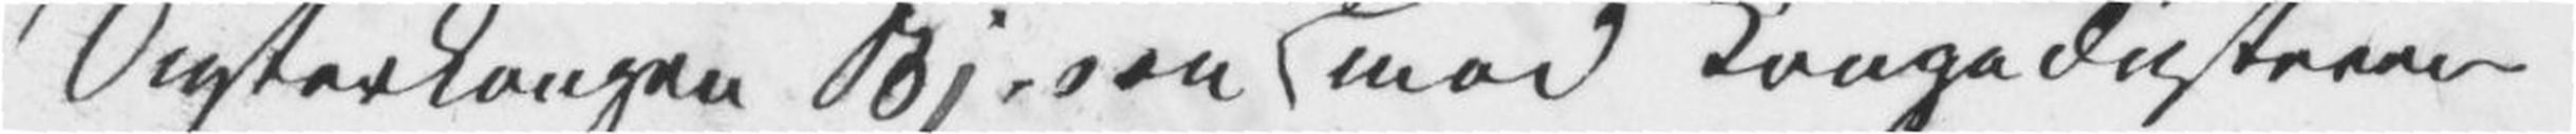Digterkongen Bj.son mod Kongedigteren
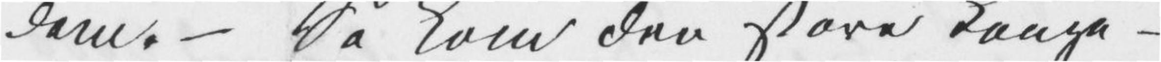dem. – Så kom den store Konge¬
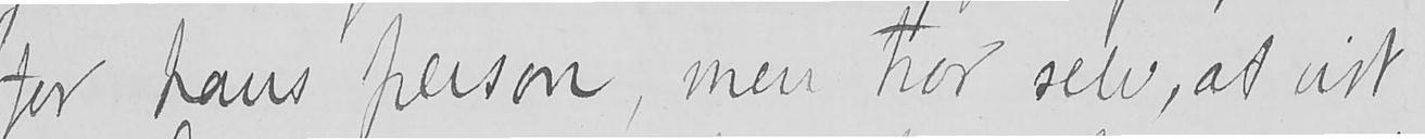for hans person, men tror selv, at vist
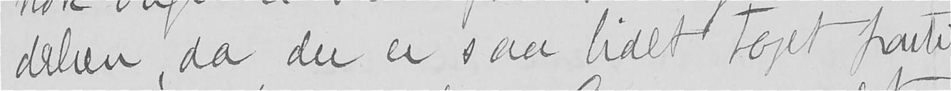delsen, da der er saa lidet taget parti
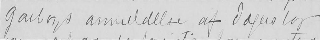Garborgs anmeldelse af Jægers bog
